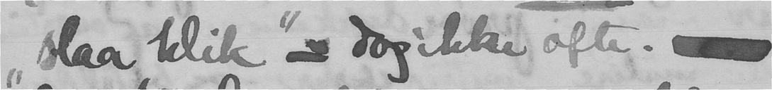"slaa klik" - dog ikke ofte. -
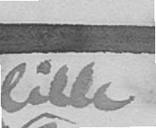lille
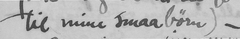til mine smaa børn) -
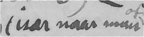(især naar man
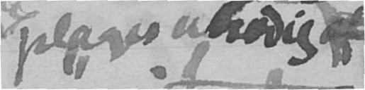plages unødig af
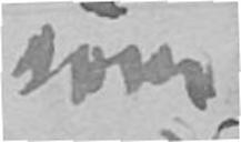som
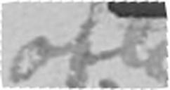ofte
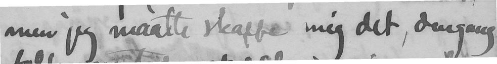men jeg maatte skaffe mig det, dengang
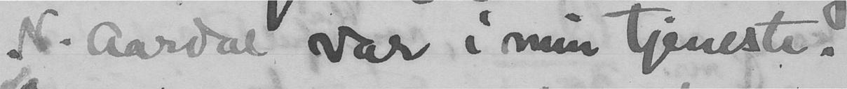N. Aardal var i min tjeneste.
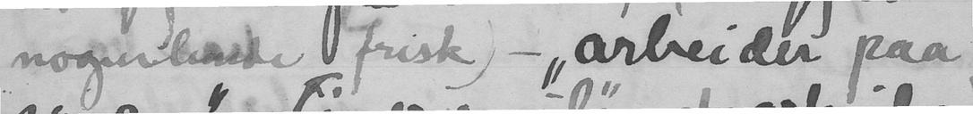nogenlunde frisk) - "arbeider paa
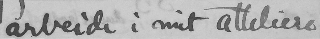arbeide i mit atteliere
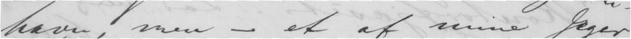havn, men - et af mine Jeger
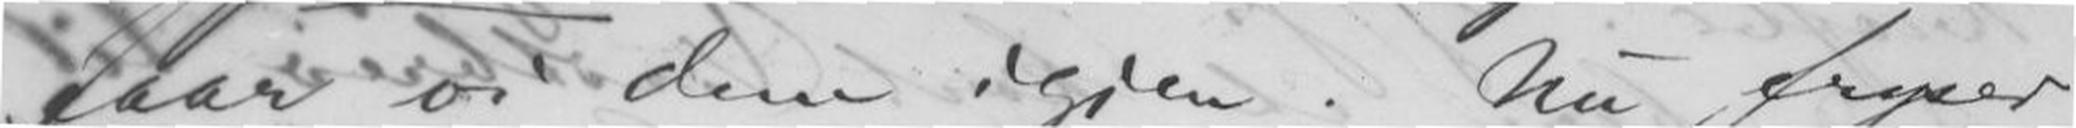Naar faar vi dem igjen. Nu fryser
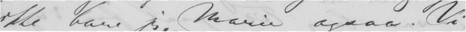ikke bare jeg, Marie ogsaa. Vi
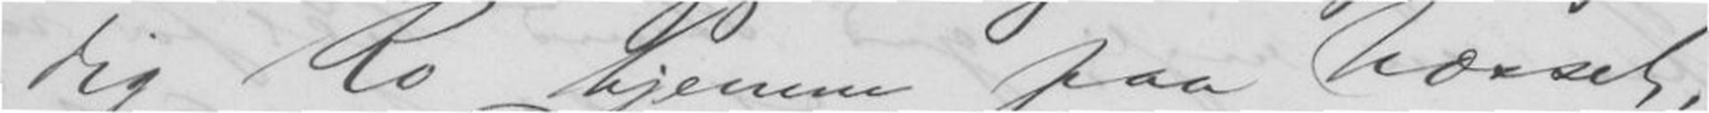dig Ro hjemme paa Næsset,
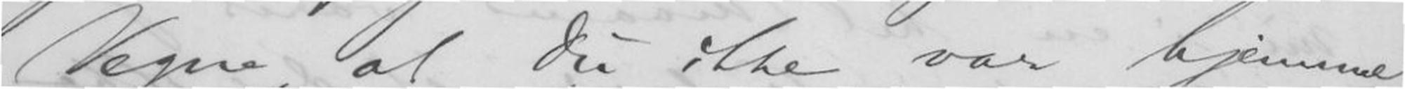Vegne, at Du ikke var hjemme,
